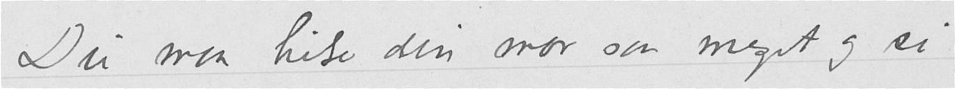Du maa hilse din mor saa meget og si
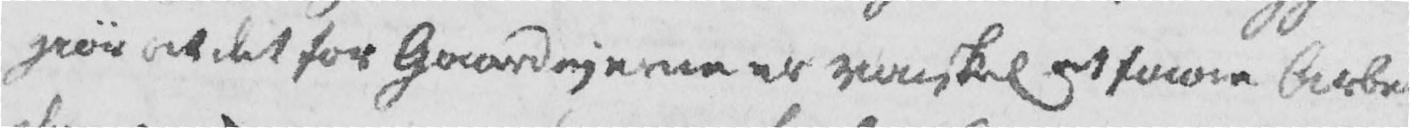giør at det for Gaardejerne er vanskel at faae Arbei-
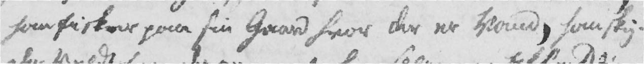han fisker paa sin Gaard hvor der er Vand, han sky-
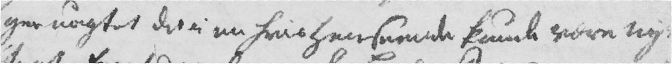ger uagtet det i en hvis Henseende kunde være rig-
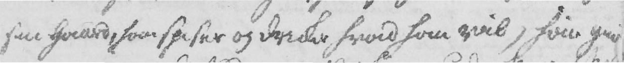sin Gaard, han spiser og dricker hvad han vil, han giør
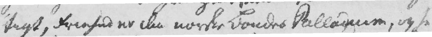tigt, Friehed er den norske Bondes Palladium, og he-
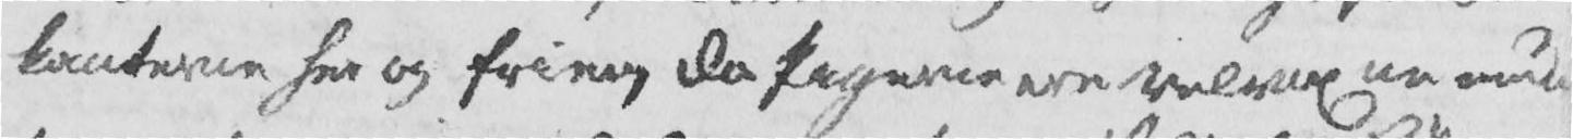kanterne her og frier da Pigerne ere velvoxne mun-
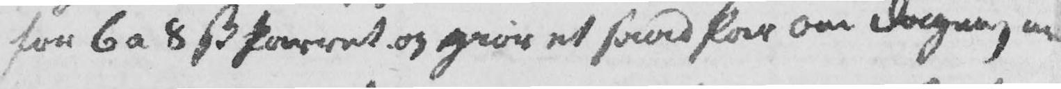for 6 a 8 sk Parret og giør et saad Par om dagen, m
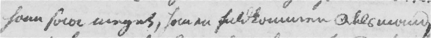ham saa meget, som en fuldkommen Adelsmand,
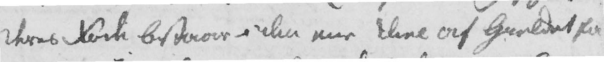Deres Føde bestaaer i den ene Deel af Gieldet for
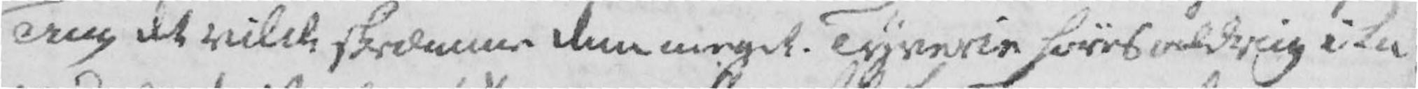Ting det vilde skræmme dem meget. Tyverie høres aldrig i Su-
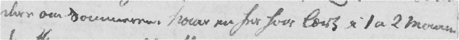dere om Sommeren. Naar en her har lært i 1 a 2 Maane-
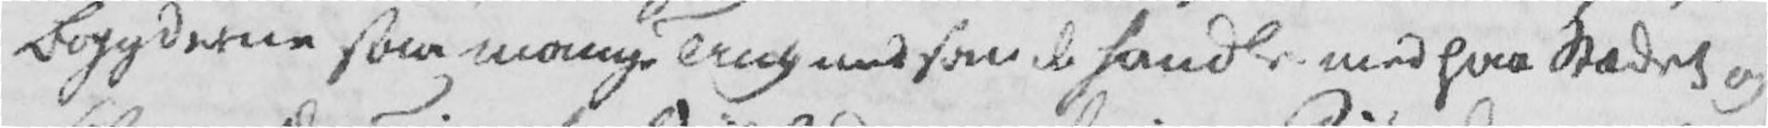Bøygderne saa mange Ting med som de handle med paa Stædet og
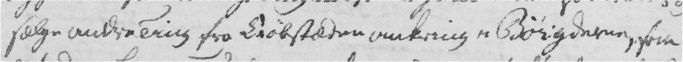sælge andre Ting fra Kiøbstædene omkring i Bøygderne, som
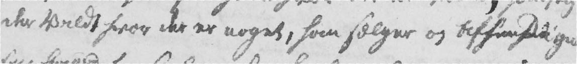der Vildt hvor der er noget, han sælger og afhændiger
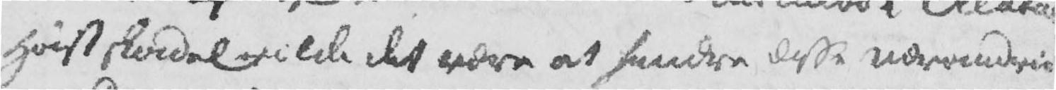Høist skadel vilde det være at hindre disse Udvandrin-
